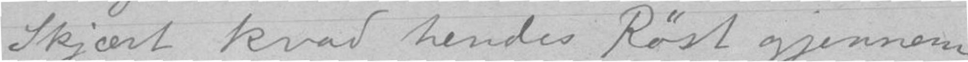Skjært kvad hendes Røst gjennem
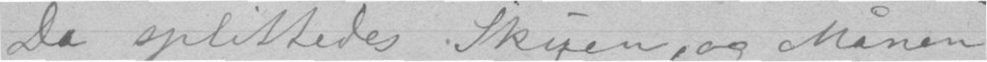Da splittedes Skyen, og Månen
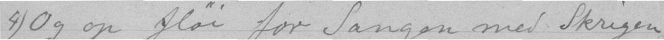4) Og op fløi for Sangen med Skrigen
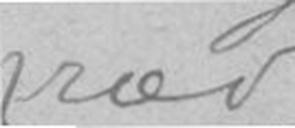ræd
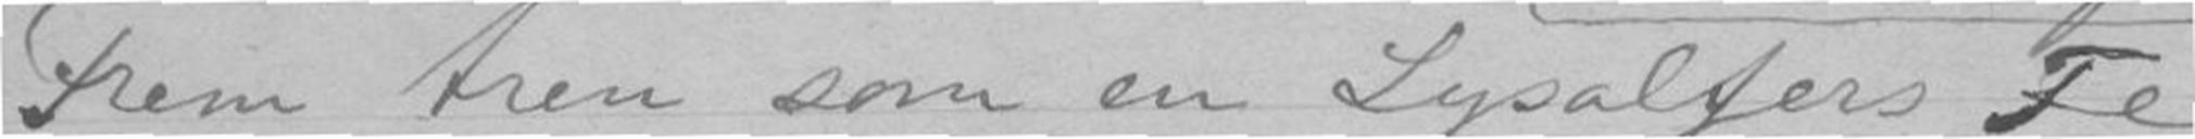Frem tren som en Lysalfers Fe
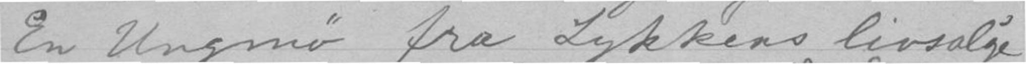En Ungmø fra Lykkens livsal'ge
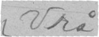Vrå
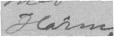Harm,
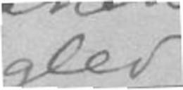gled
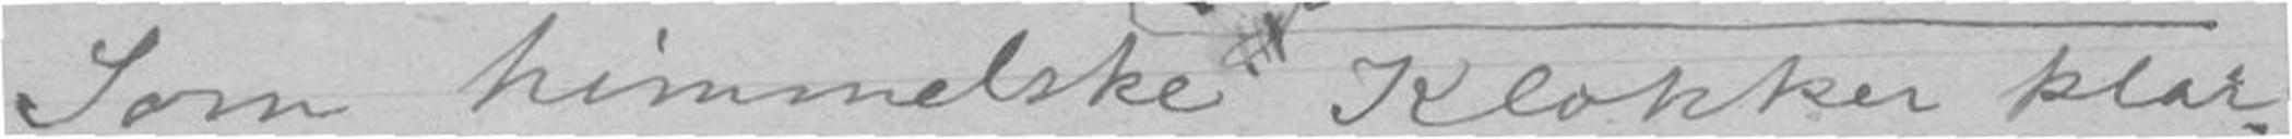Som himmelske Klokker klar.
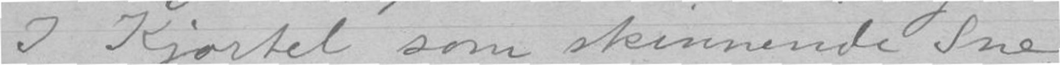I Kjørtel som skinnende Sne.
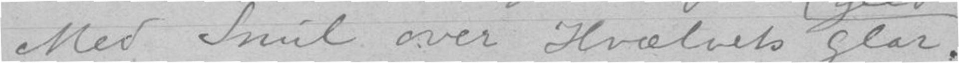Med Smil over Hvælvets Glar.
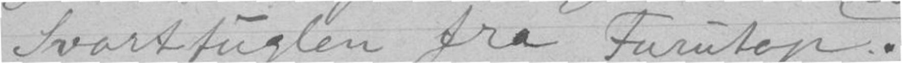Svartfuglen fra Furutop.
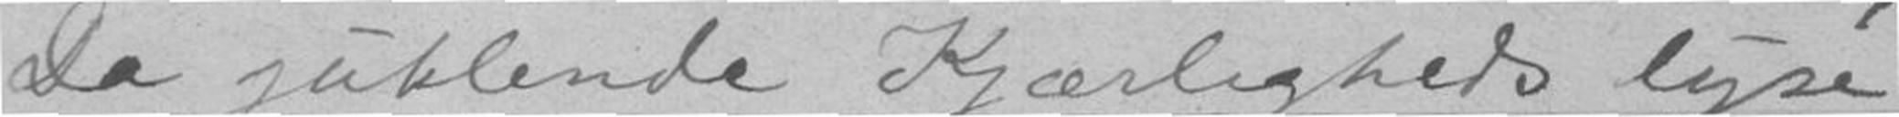Da jublende Kjærligheds lyse
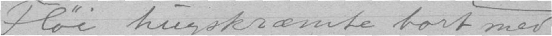Fløi hugskræmte bort med
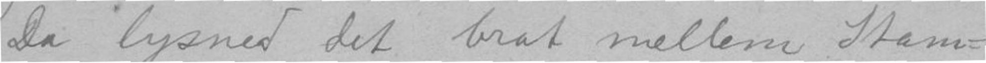3) Da lysned det brot mellem Stam-
